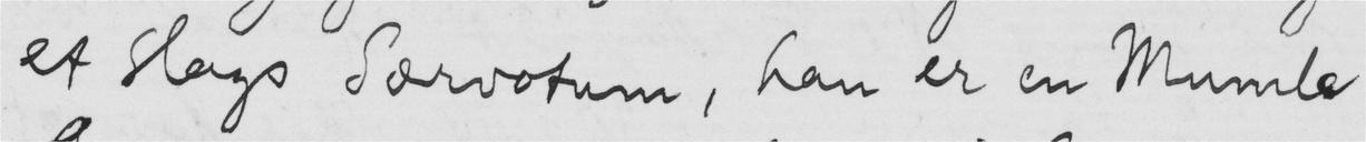et slags Særvotum, han er en Mumle
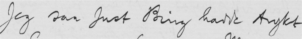Jeg saa Just Bing hadde trykt
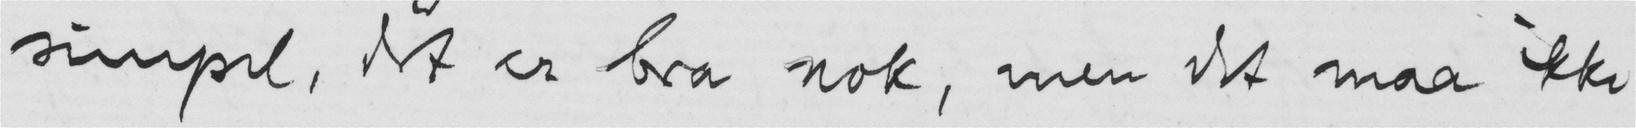simpel, det er bra nok, men det maa ikke
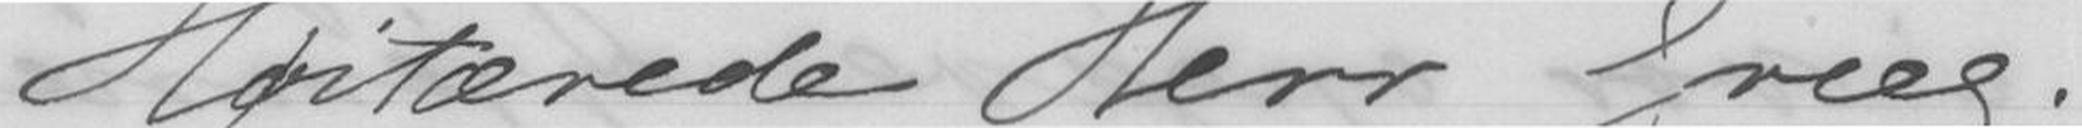Høystærede Herr. Grieg.
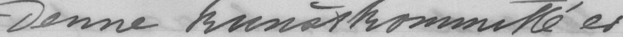Denne kunstkommitté er
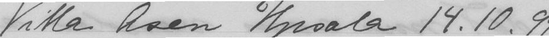Villa Åsen Upsala 14.10.99
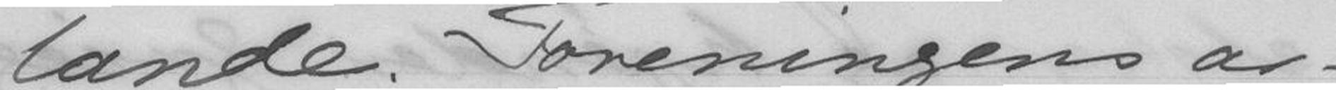lande. Foreningens ar-
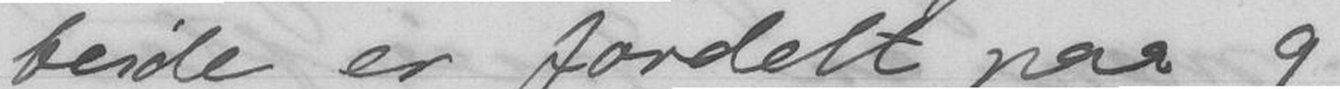beide er fordelt paa 9
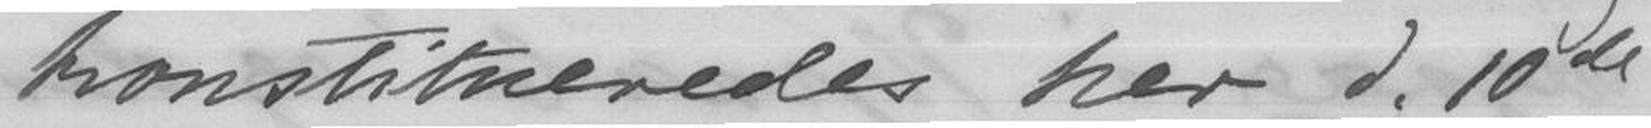konstitueredes her d.10de
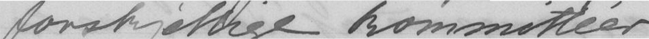forskjellige kommittéer
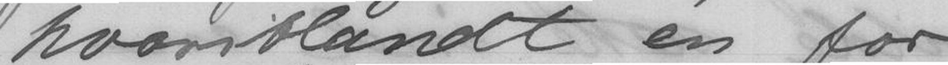hvoriblandt én for
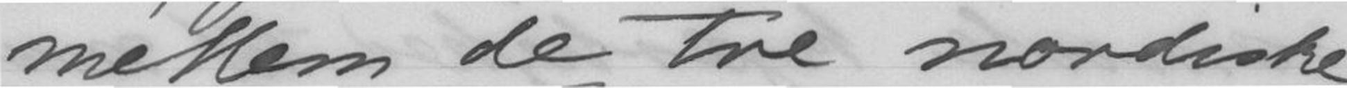mellem de Tre nordiske
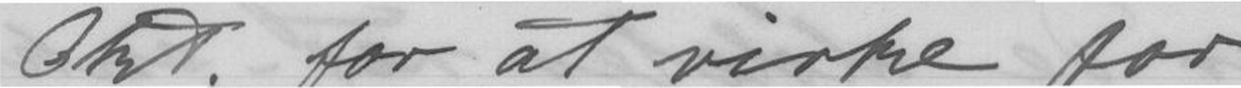Okt. for at virke for
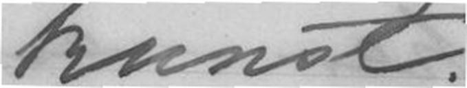kunst.
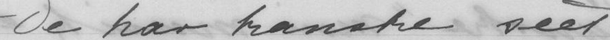De har kanske seet
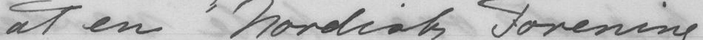at en "Nordisk Forening"
